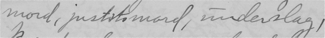mord, justismord, underslag,
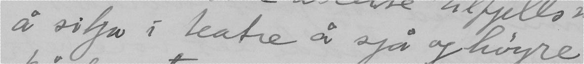å sitja i teatre å sjå og höyre
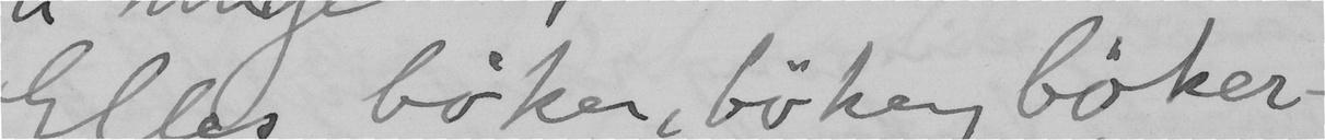Elles böker, böker, böker.
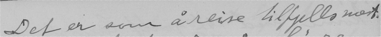Det er som å reise tilfjells nært.
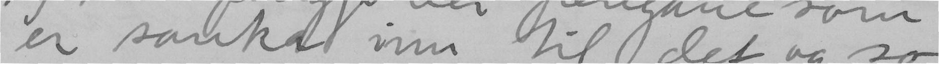er sanka inn til det og so
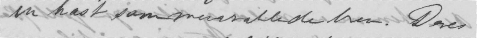en hast sammenrablede brev. Deres
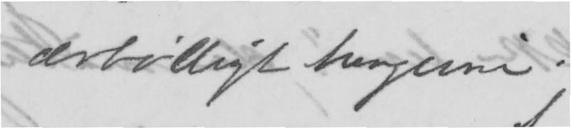ærbødigt hengivne.
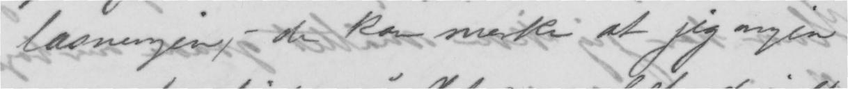læsningen, de kan merke at jeg ingen
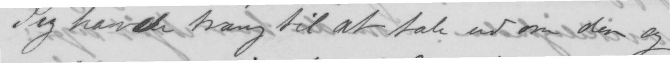Jeg havde trang til at tale ud om den og
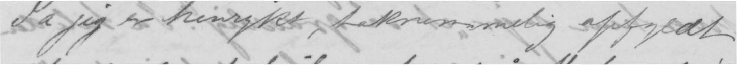Ja jeg er henrykt, taknemmelig opfyldt
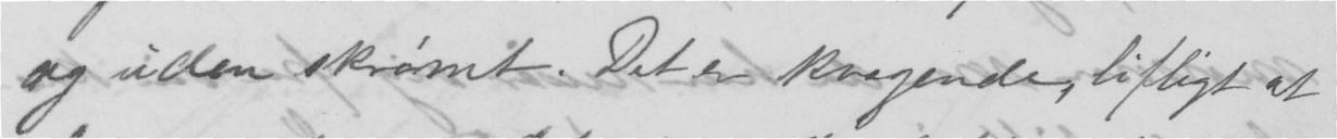og uden skrømt. Det er kvægende, lifligt at
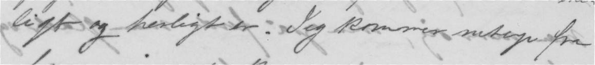ligt og herligt er. Jeg kommer netop fra
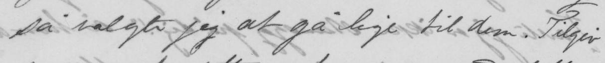så valgte jeg at gå lige til dem. Tilgiv
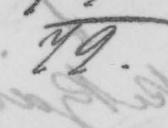79.
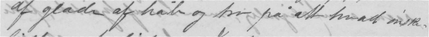af glæde af håb og tro på alt hvad ønske-
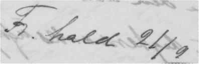Fr. hald 21/9
